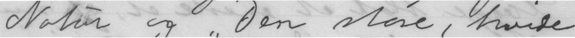Natur og "Den store, hvide
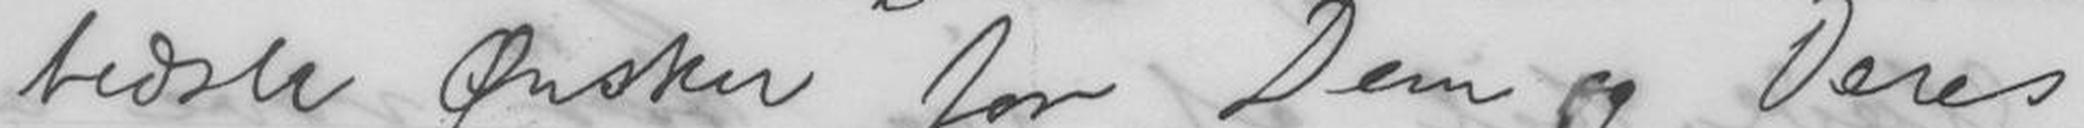bedste Ønsker for Dem og Deres
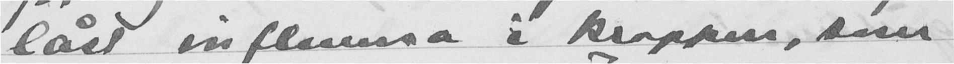låst influensa i kroppen, som
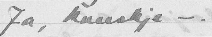Ja, kanskje -.
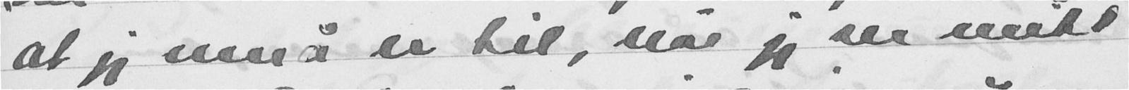at jeg ennå er til, når jeg ser mitt
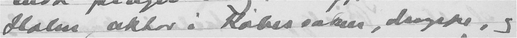Holm, aktor i Kober saken, deroppe, og
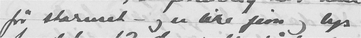før stormet - og er like jevn og lys
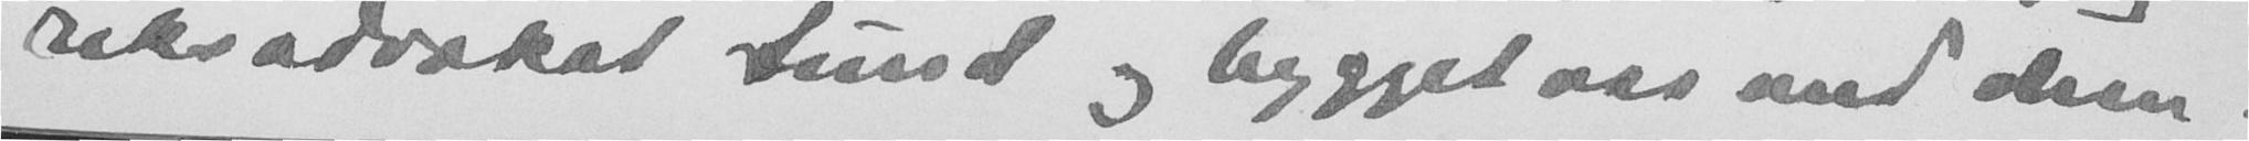riksadvokat Sund og hygget oss med dem.
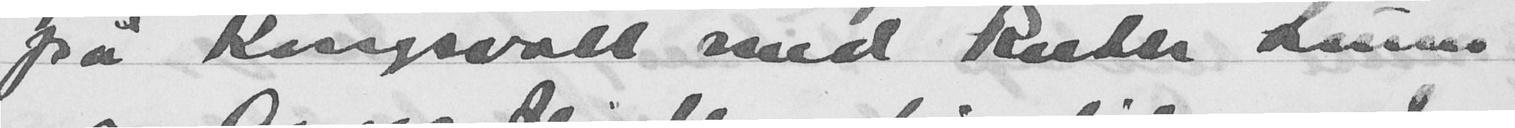på Kongsvoll med Ruth Leum
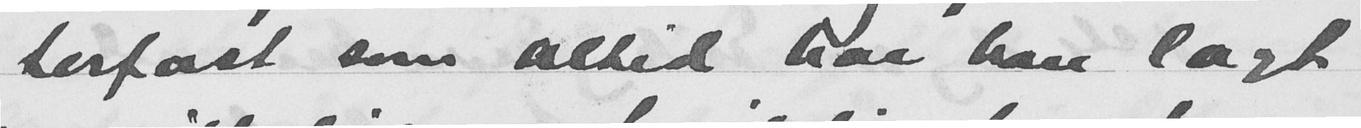terfast som altid har han lagt
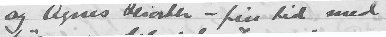og Agnes Hiorth - fin tid med
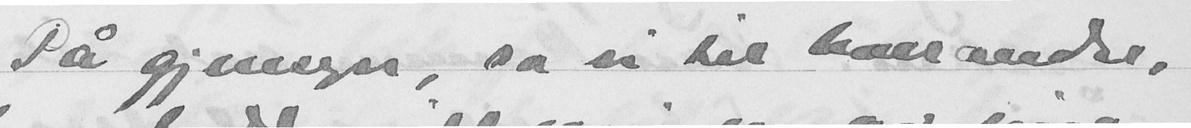På gjensyn, sa vi til hverandre,
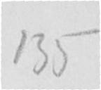135
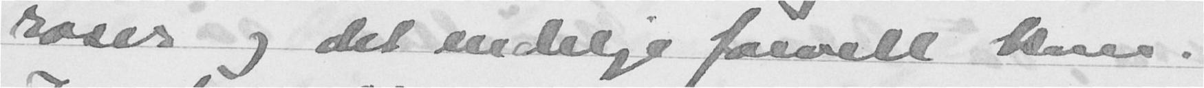roser og det endelige farvell kom.
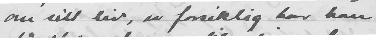om sitt liv, er forsiktig hvor han
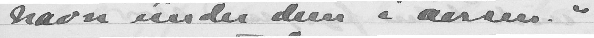navn under dem i avisen."
